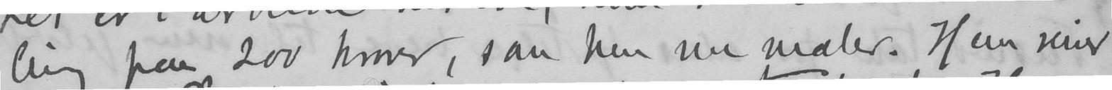ling paa 200 kroner, som hun nu maler. Hun reiser
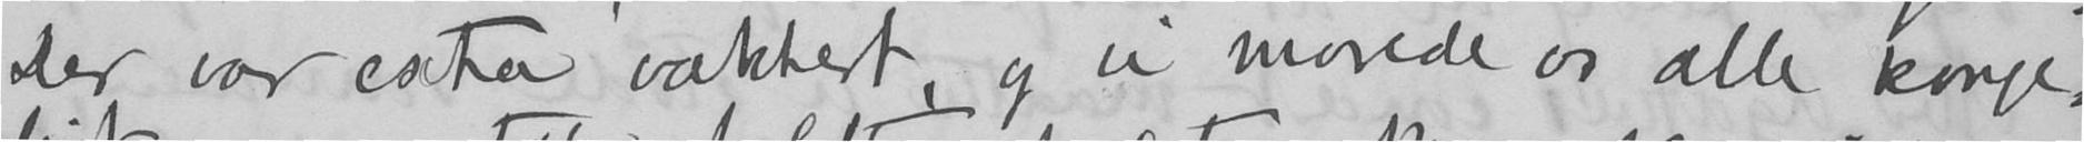Der var extra vakkert, og vi morede os alle konge-
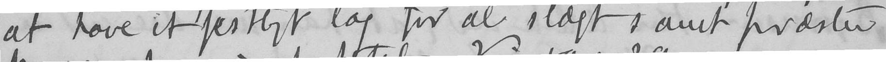at have et festligt lag for al slægt samt præsten
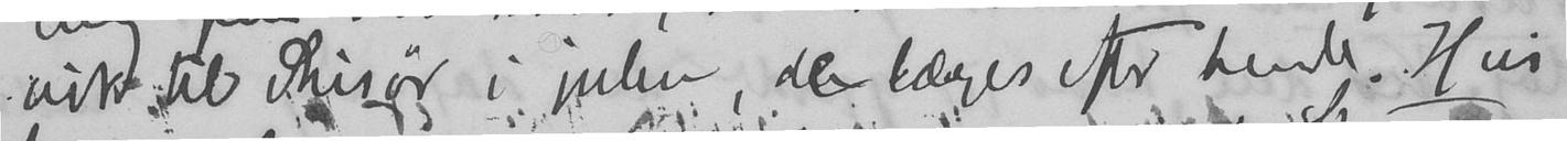vist til Risør i julen, de længes efter hende. Hvis
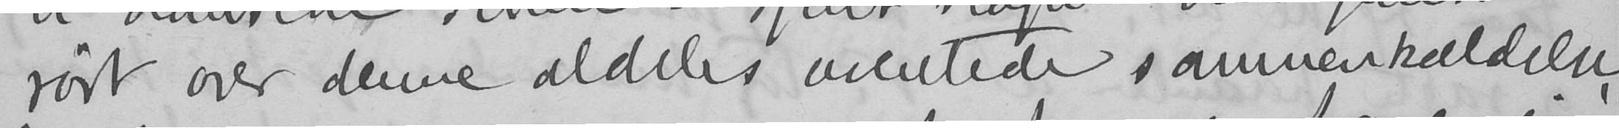rørt over denne aldeles uventede sammenkaldelse,
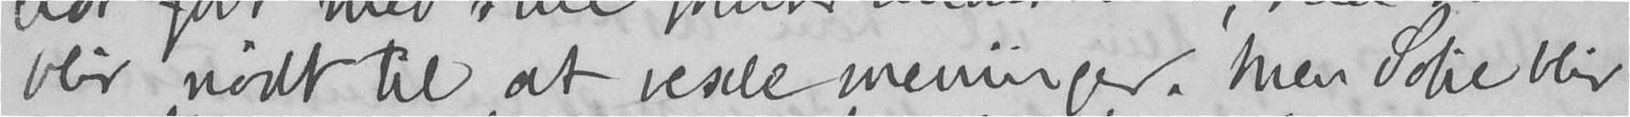blir nødt til at vexle meninger. Men Sofie blir
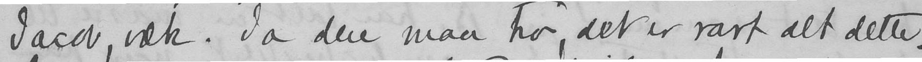Jacob, væk. Ja dere maa tro, det er rart alt dette.
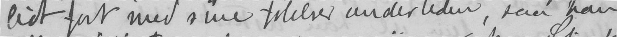lidt fort med sine følelser undertiden, saa han
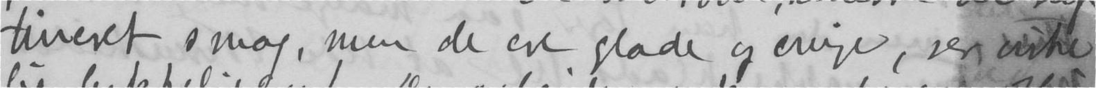fineret smag, men de ere glade og enige, ser virke-
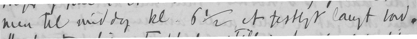men til middag kl. 6½ et festligt langt bord.
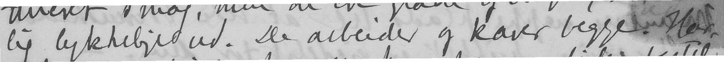lig lykkelige ud. De arbeider og kaver begge. Har-
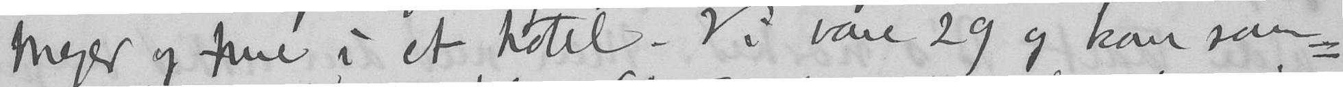Meyer og frue i et hotel. Vi vare 29 og kom sam-
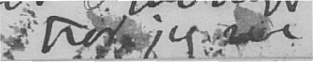tror jeg sel
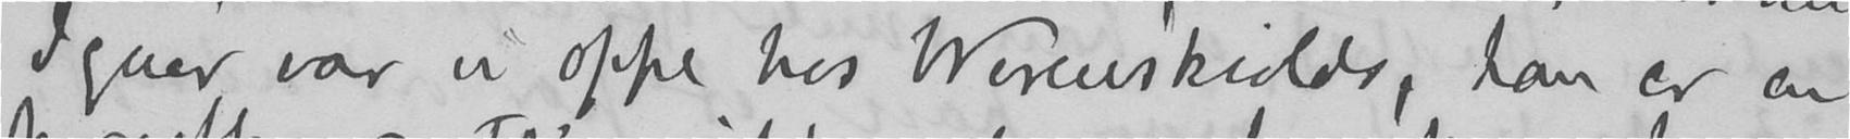Igaar var vi oppe hos Werenskiolds, han er en
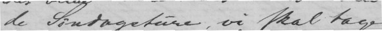de Søndagsture, vi skal tage
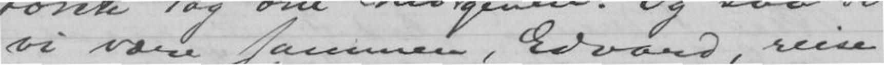vi være sammen, Edvard, reise
